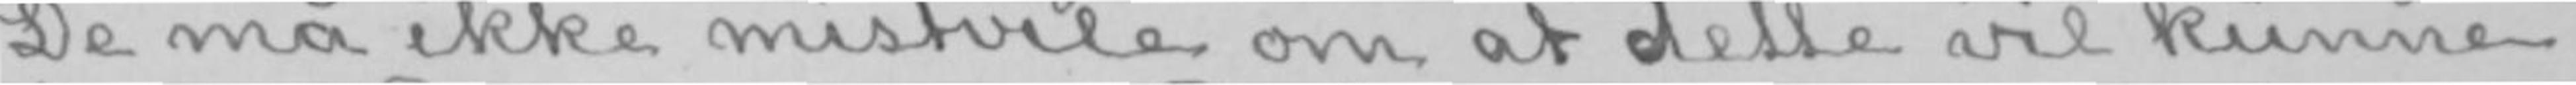De må ikke mistvile om at dette vil kunne
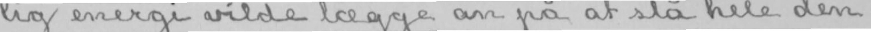lig energi vilde lægge an på at slå hele den
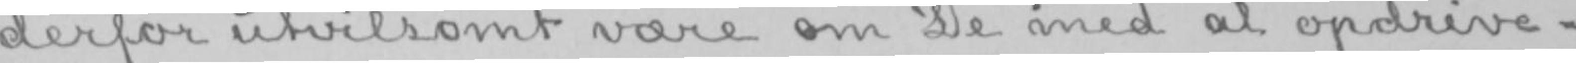derfor utvilsomt være om De med al opdrive-
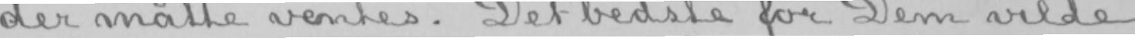der måtte ventes. Det bedste for Dem vilde
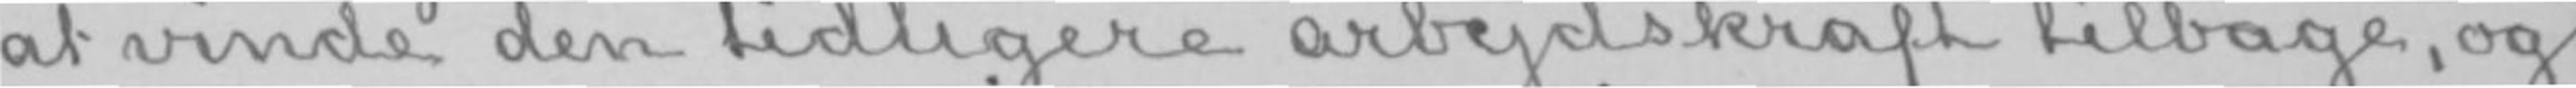at vinde den tidligere arbejdskraft tilbage, og
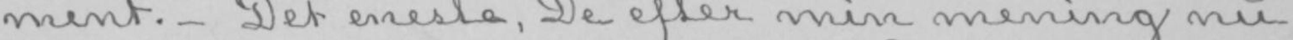ment.- Det eneste, De efter min mening nu
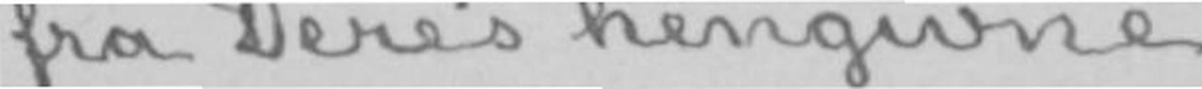fra Deres hengivne
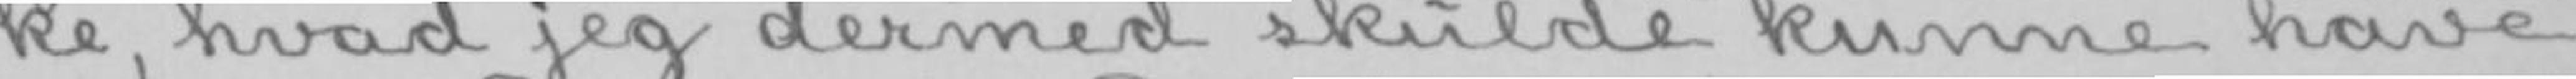ke, hvad jeg dermed skulde kunne have
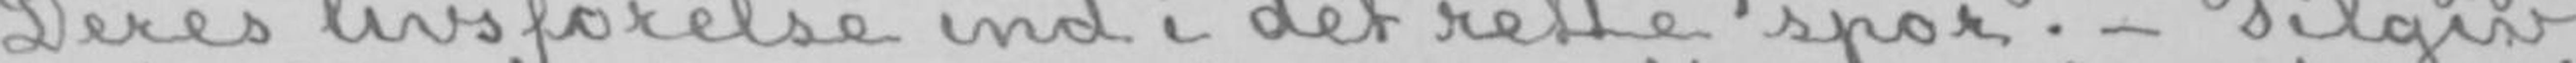Deres livsførelse ind i det rette spor.- Tilgiv
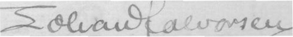Johan Halvorsen
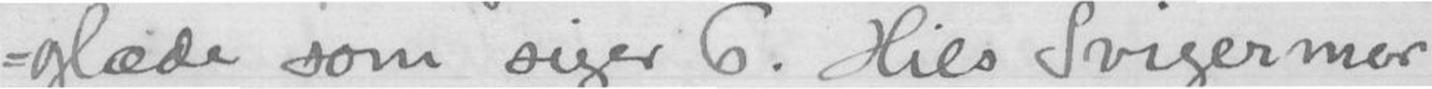glæde som siger 6. Hils Svigermor
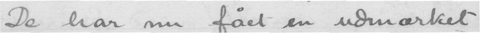De har nu fået en udmærket
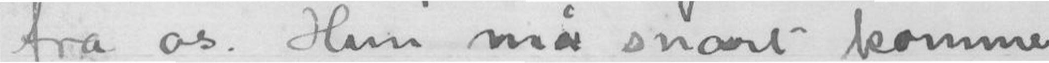fra os. Hun må snart komme
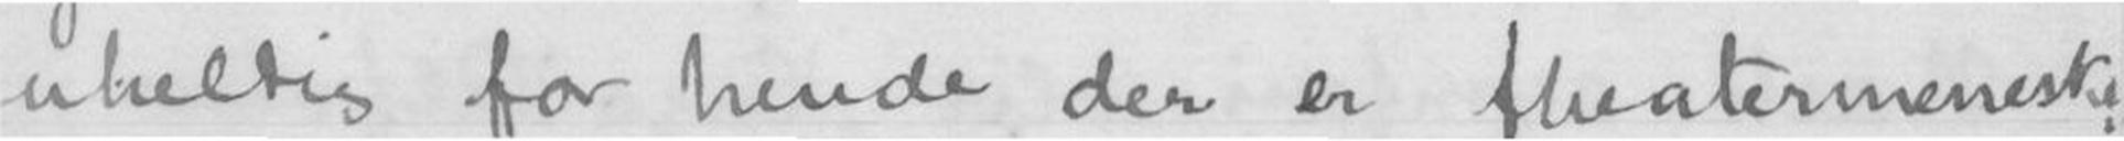uheldig for hende der er theatermeneske.
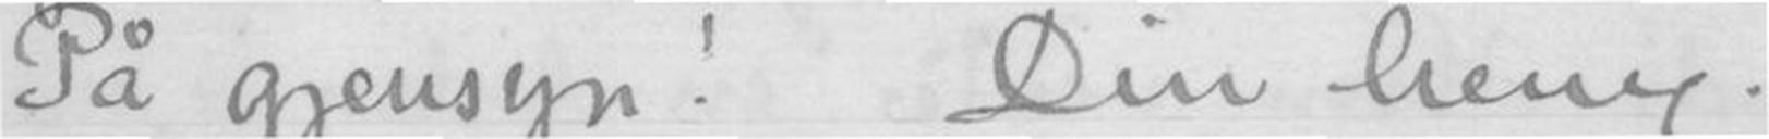På gjensyn! Din heng.
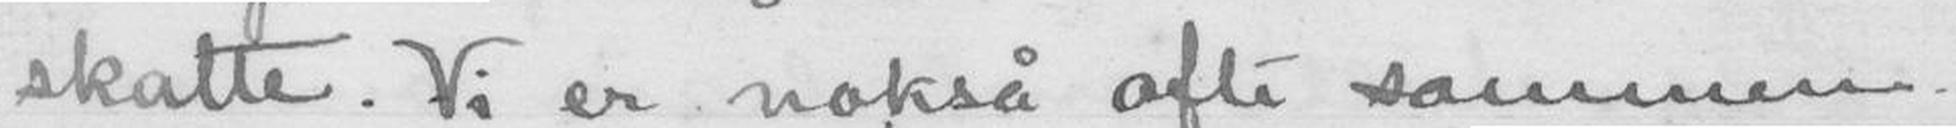skatte. Vi er nokså ofte sammen.
